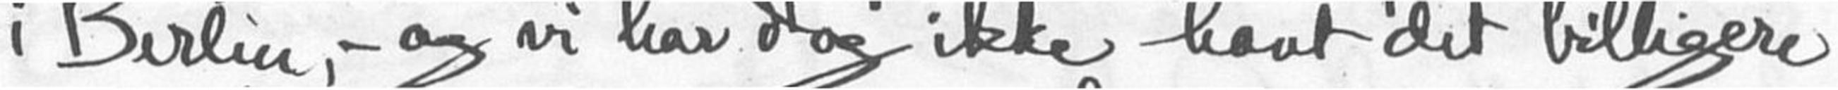i Berlin, - og vi har dog ikke havt det billigere
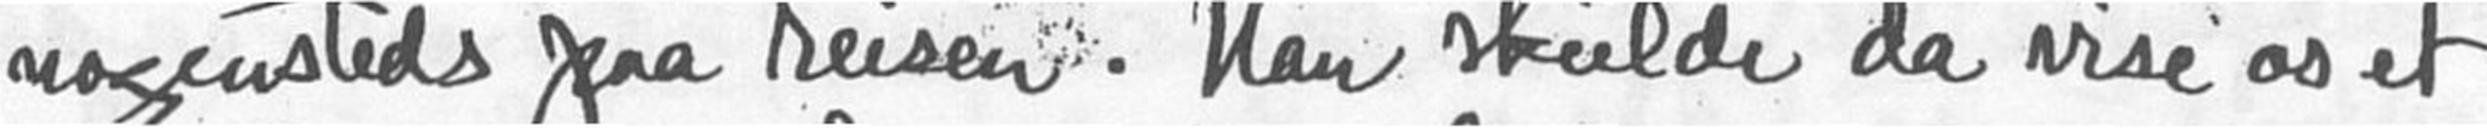nogensteds paa reisen. Han skulde da vise os et
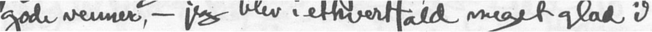gode venner, - jeg blev i ethvertfald meget glad i
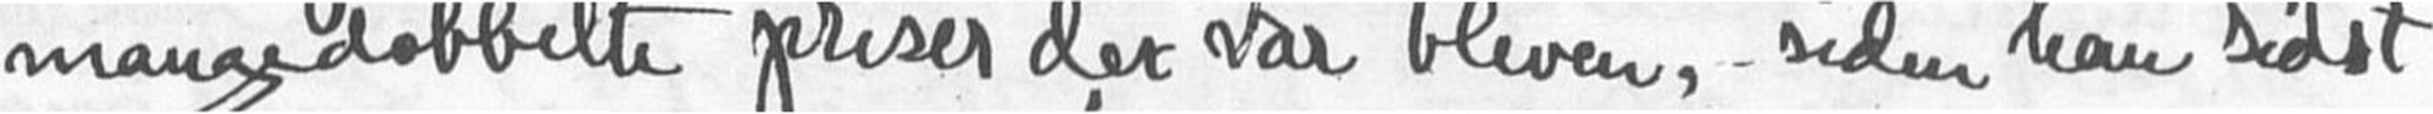mangedobbelte priser det var bleven,- siden han sidst
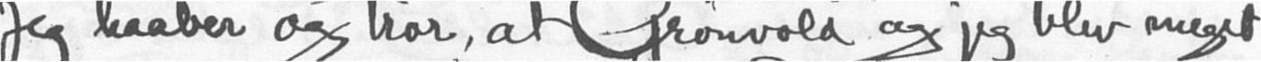Jeg haaber og tror, at Grønvold og jeg blev meget
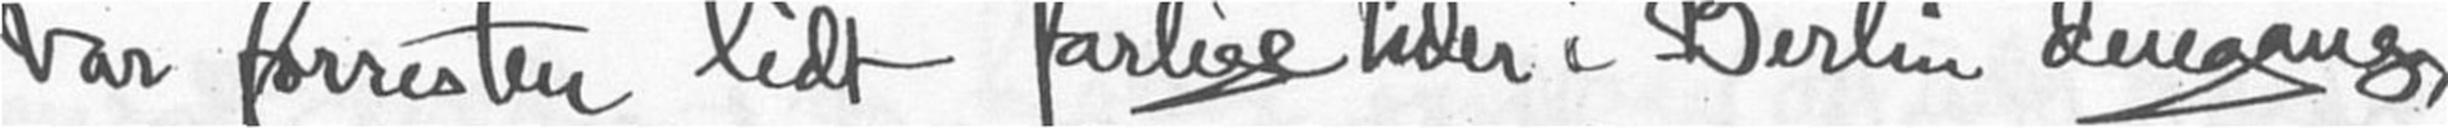var forresten lidt farlige tider i Berlin dengang,
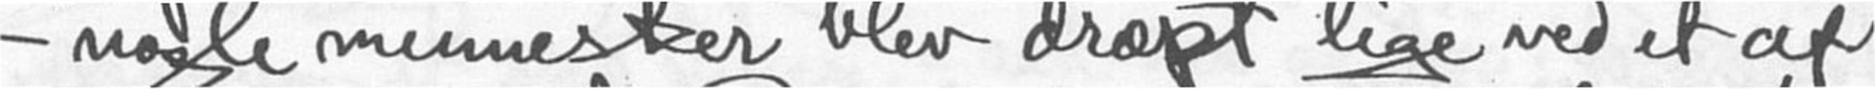- nogle mennesker blev dræpt lige ved et af
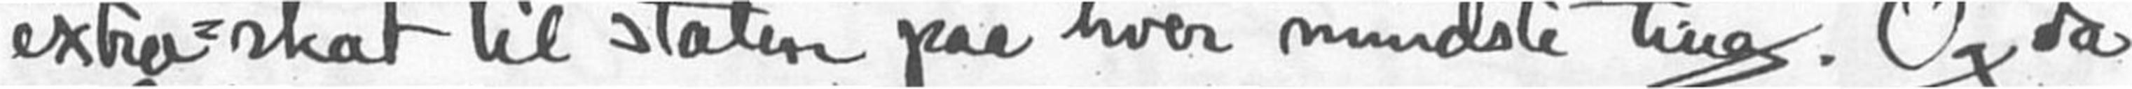extra-skat til staten paa hver mindste ting. Og da
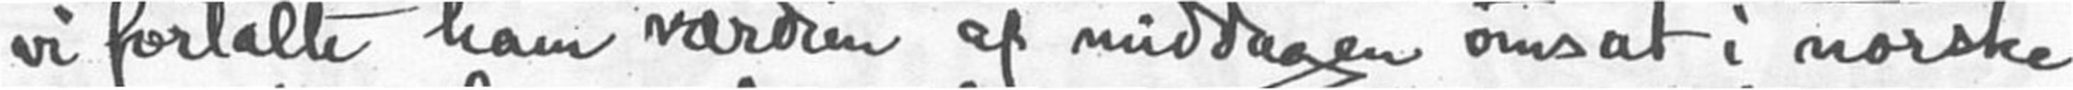vi fortalte ham værdien af middagen omsat i norske
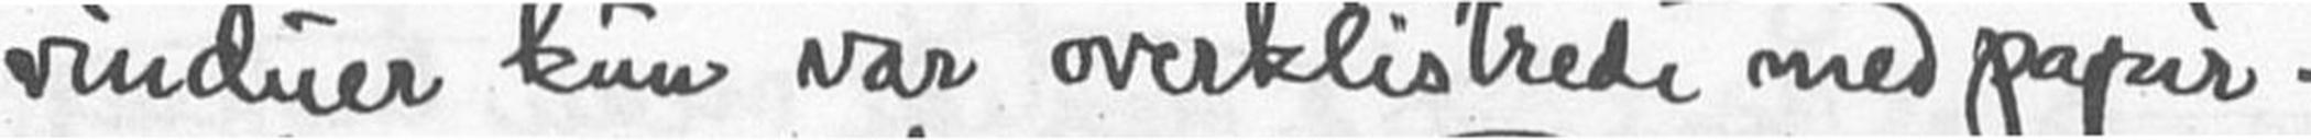vinduer kun var overklistrede med papir. -
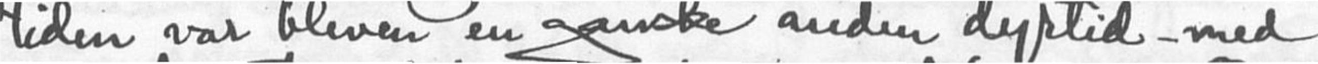tiden var bleven en ganske anden dyrtid - med
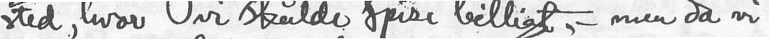sted, hvor vi skulde spise billigt,- men da vi
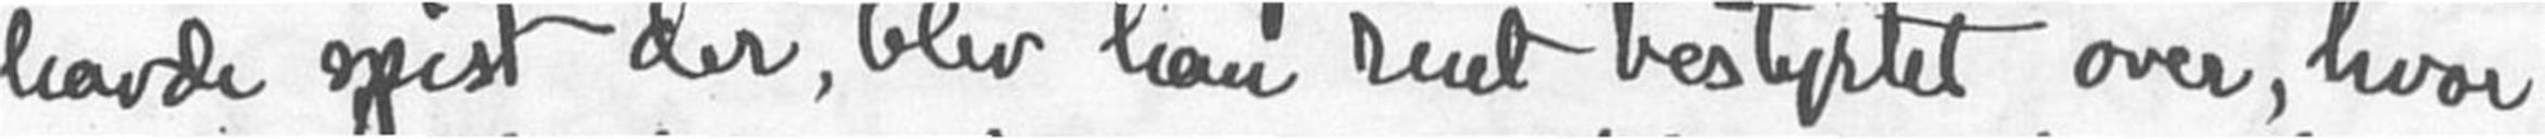havde spist der, blev han rent bestyrtet over, hvor
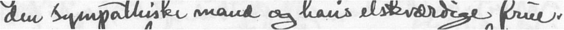den sympathiske mand og hans elskværdige frue.
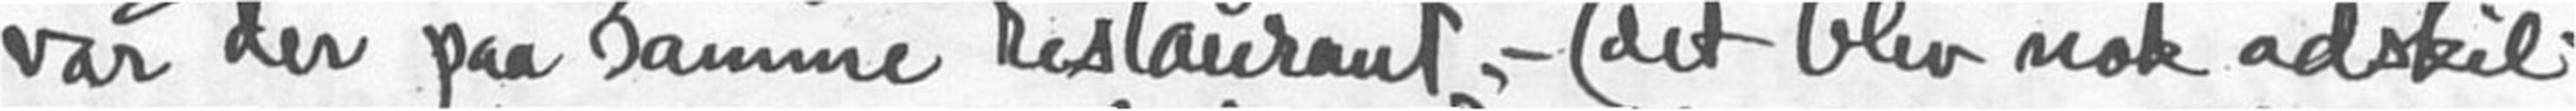var der paa samme restaurant,- (det blev nok adskil-
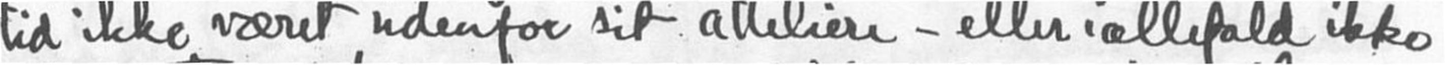tid ikke været udenfor sit attelier - eller iallefald ikke
